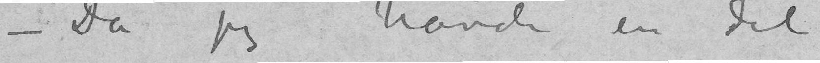– Da jeg havde en del
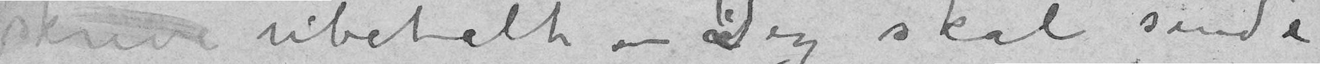skuve ubetalt – Jeg skal sende
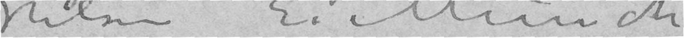Hilsen E. Munch
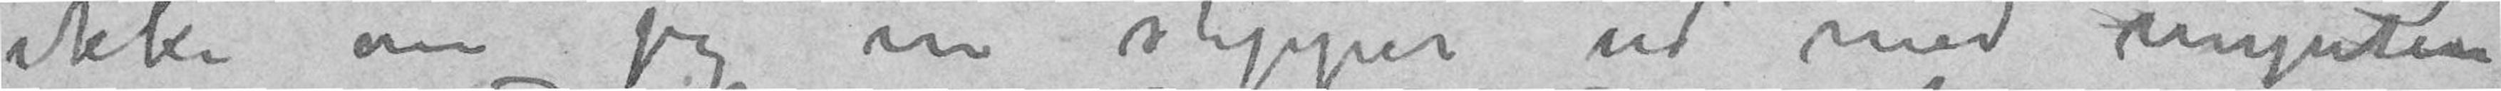ikke om jeg nu slipper ud med mynten
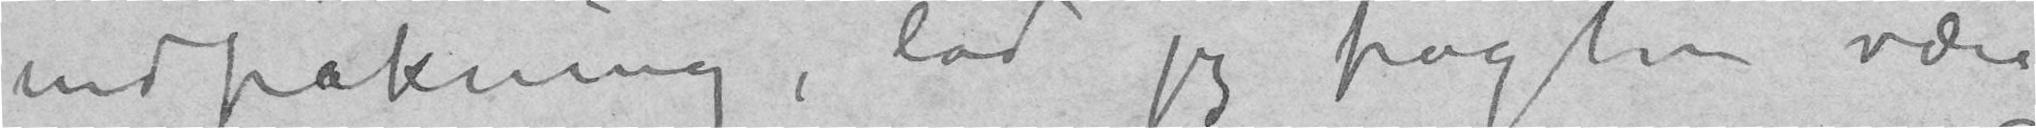indpakning, lod jeg fragten være
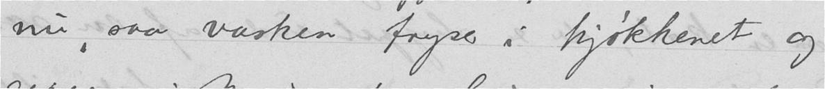nu, saa vasken fryser i kjøkkenet og
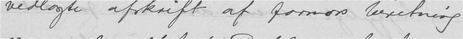vedlagte afskrift af farmors beretning
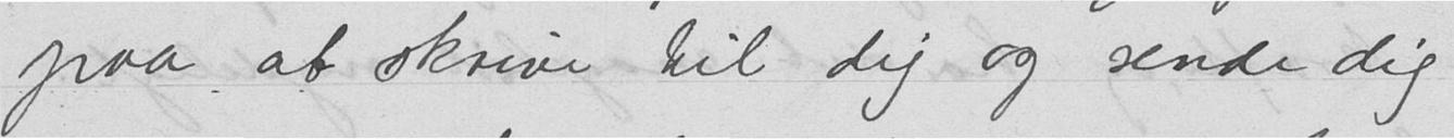paa at skrive til dig og sender dig
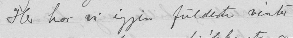Her har vi igjen fuldeste vinter
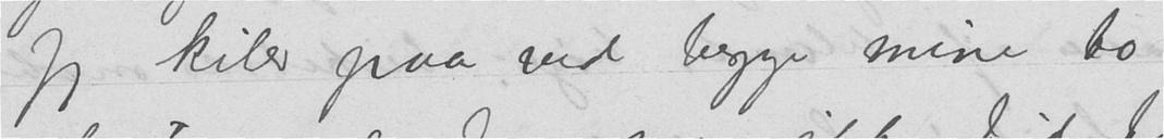Jeg kiler paa ved begge mine to
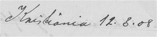Kristiania 12.8.08
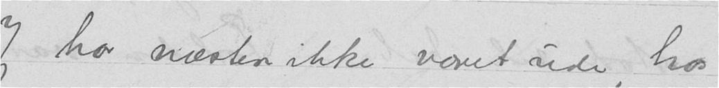Jeg har næsten ikke været ude, hos
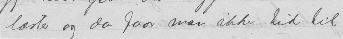læster og da faar man ikke tid til
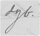dyb.
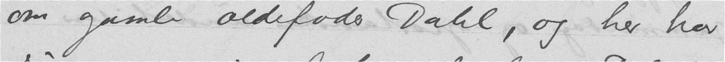om gamle oldefader Dahl, og her har
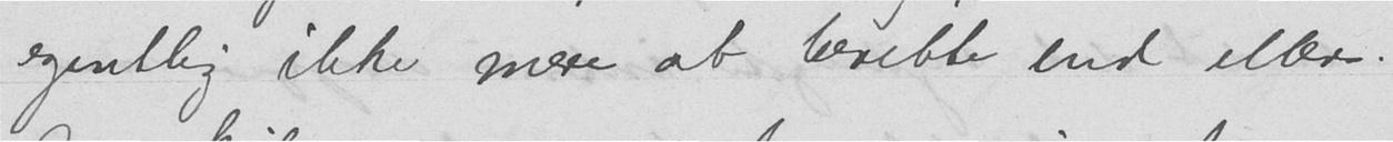egentlig ikke mere at berette end ellers.
